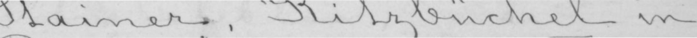Stainer, Kitzbüchel in
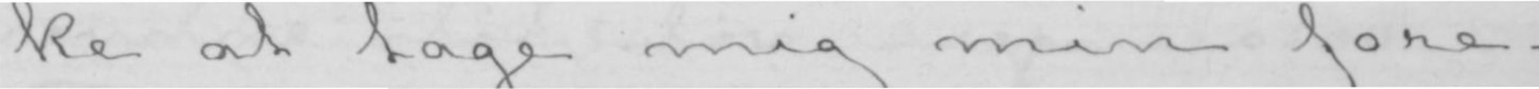ke at tage mig min fore-
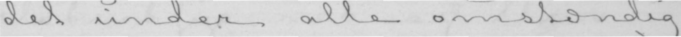det under alle omstændig-
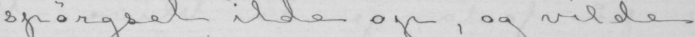spørgsel ilde op, og vilde
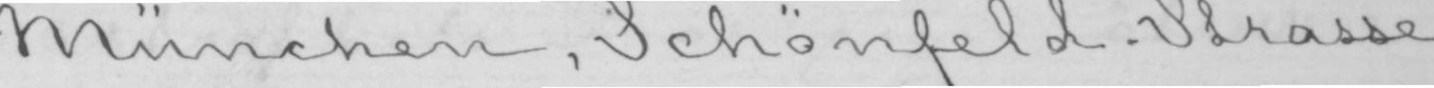München, Schönfeld-Strasse,
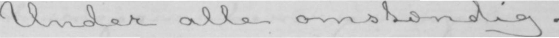Under alle omstændig-
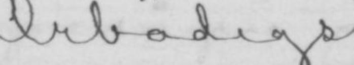Ærbødigst
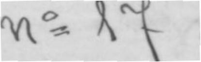No 17.
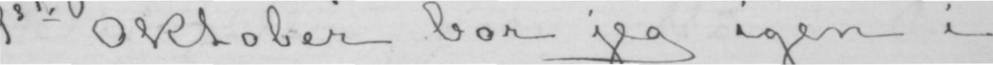1ste Oktober bor jeg igen i
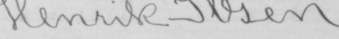Henrik Ibsen.
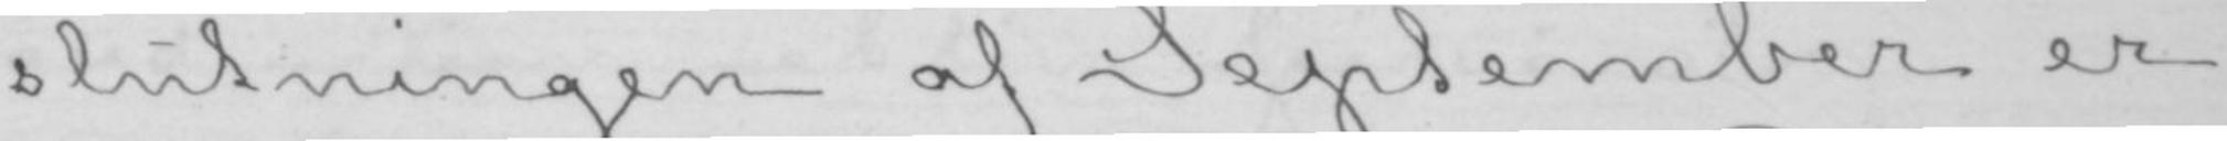slutningen af September er
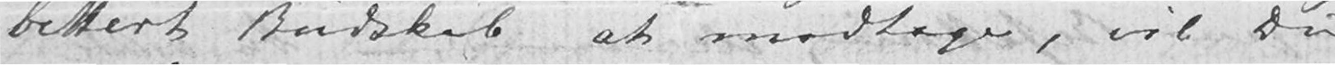bittert Budskab at modtage, vil Du
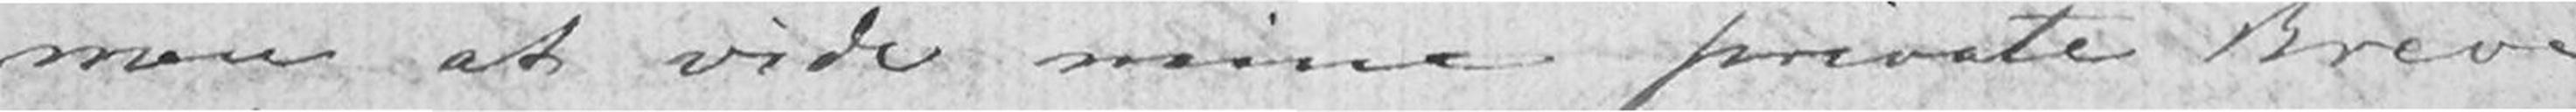men at vide mine private Breve,
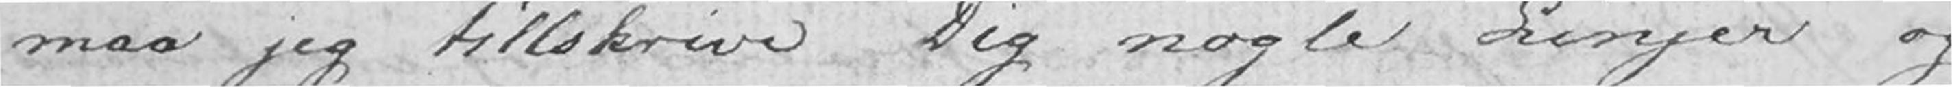maa jeg tillskrive Dig nogle Linjer og
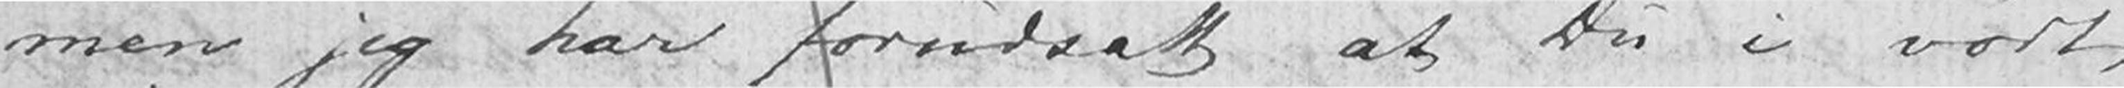men jeg har forudsatt at Du i vort
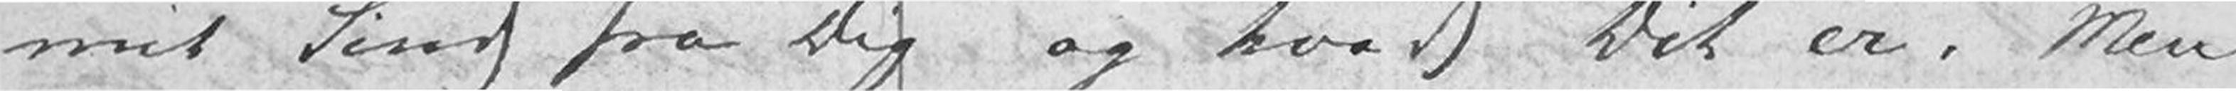mit Sind fra Dig og hvad Dit er. Men
